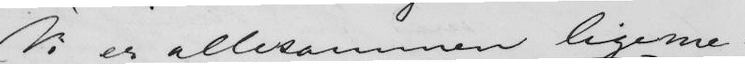Vi er allesammen ligeme-
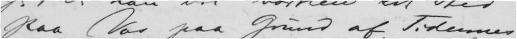paa Vos paa Grund af Tidernes
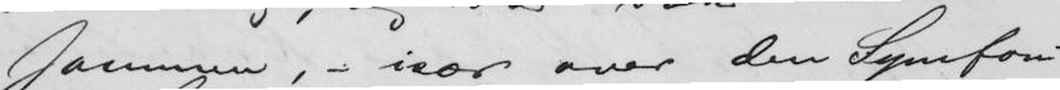sammen, - især over den Symfoni
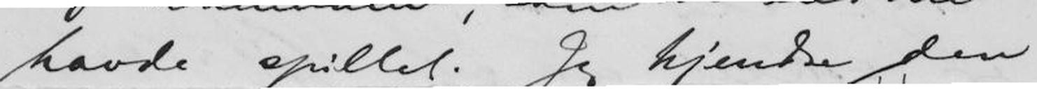havde spillet. Jeg kjendte den
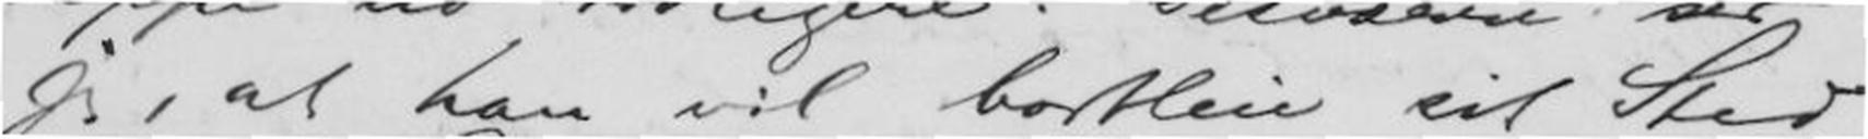jeg, at han vil bortleie sit Sted
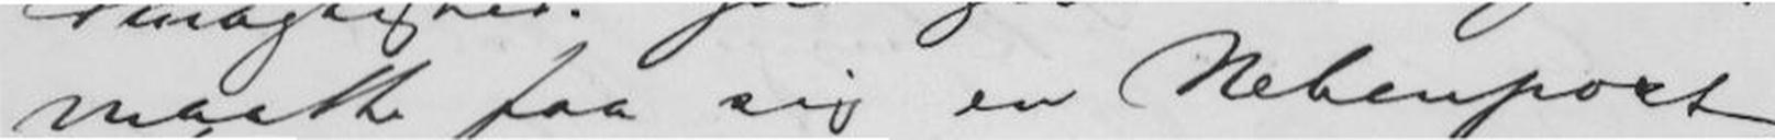maatte faa sig en Nebenpost
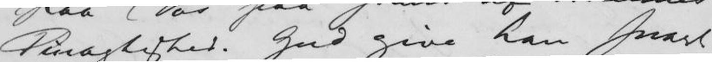Pinagtighed. Gud give han snart
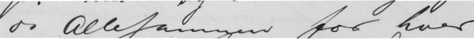os Allesammen for hver
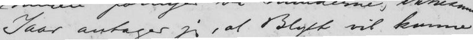Iaar antager jeg, at Blytt vil kunne
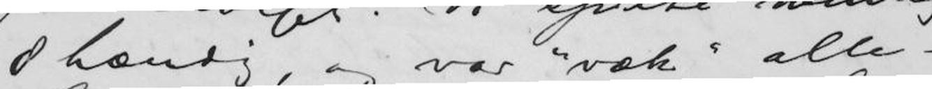8 hændig, og var "væk" alle-
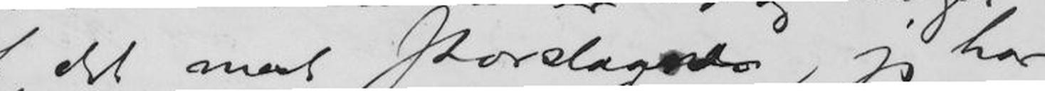af det mest storslagne, jeg har
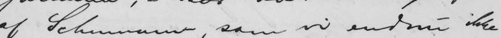af Schumann, som vi endnu ikke
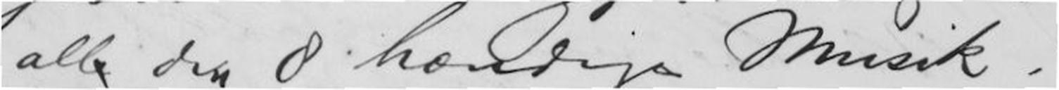all den 8 hændige Musik!
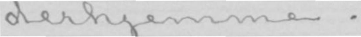derhjemme.
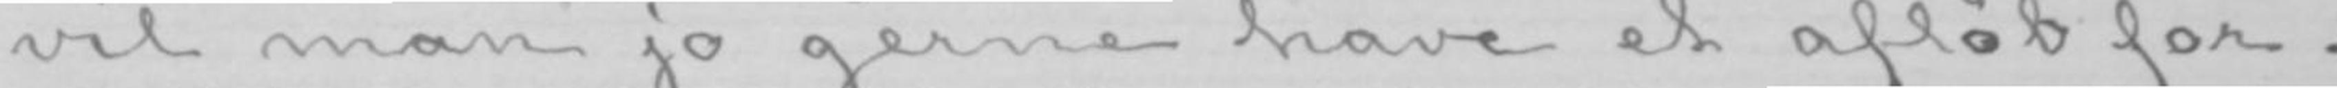vil man jo gerne have et afløb for.
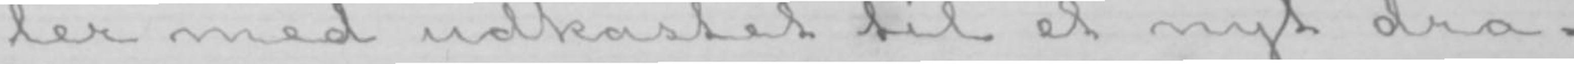ler med udkastet til et nyt dra-
